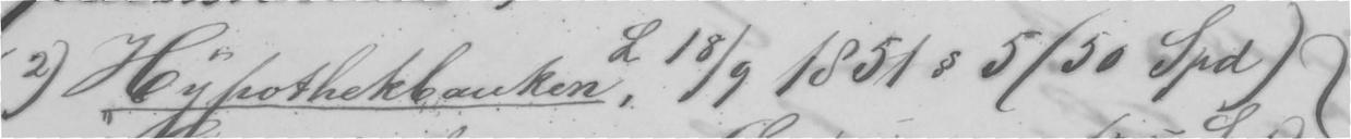2) Hypothekbanken, L 18/9 1851 § 5/50 Spd)
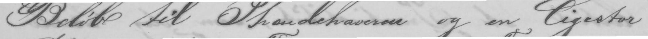Beløb til Phandehavernen og en ligestor
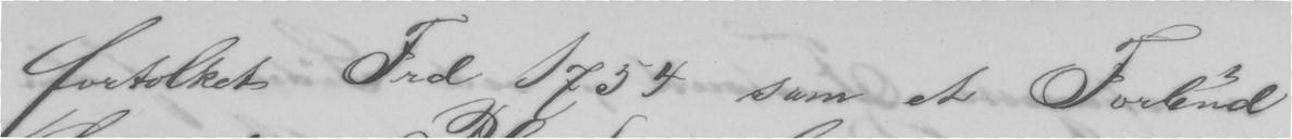fortolket i Frd 1754 som et Forbud
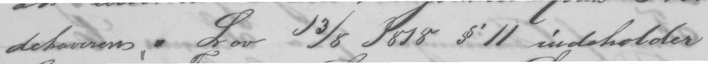dehaveren. Lov 13/8 § 11 indeholder
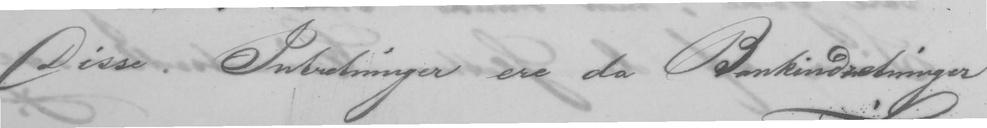Disse Inbretninger ere da Bankindretninger
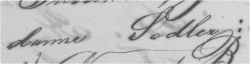danne Sedler:
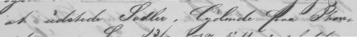at udstede Sedler, lydende paa Phan-
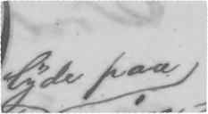lyde paa
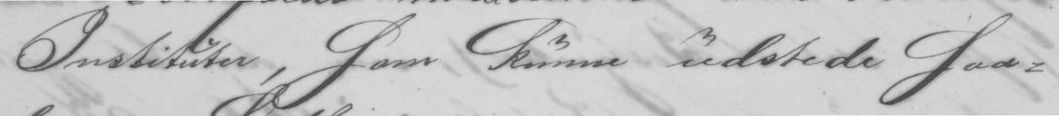Instituter, som kunne udstede saa=
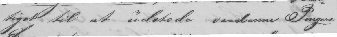tiget til at udstede saadanne Pengere
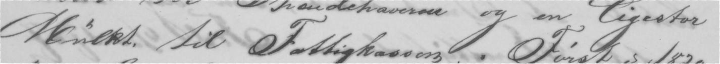Mulkt. til Fattigkassen. Først i 1820-
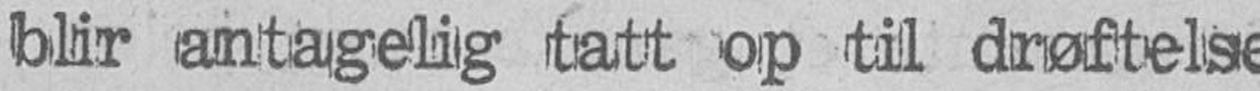blir antagelig tatt op til drøftelse
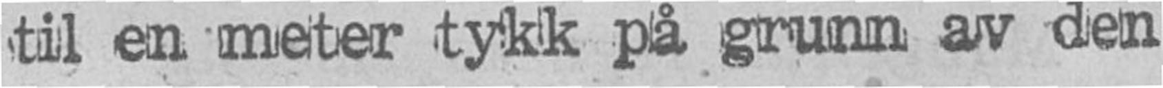til en meter tykk pà grunn av den
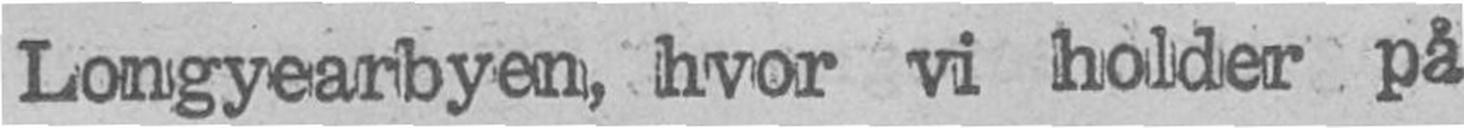Longyearbyen, hvor vi holder på
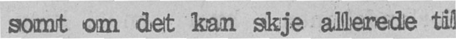somt om det kan skje allerede til
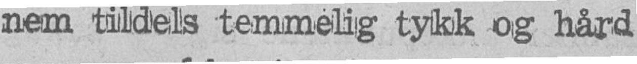nem tildels temmelig tykk og hård
